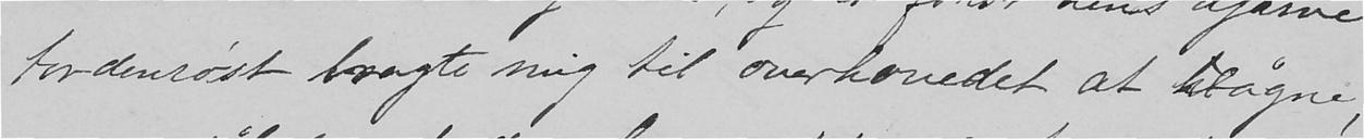tordenrøst bragte mig til overhovedet at vågne
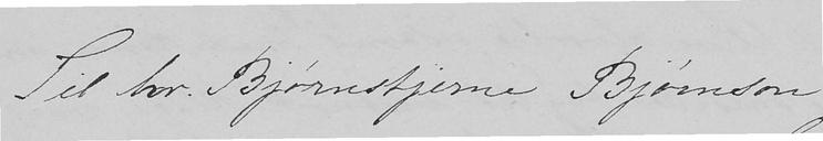Til hr. Bjørnstjerne Bjørnson
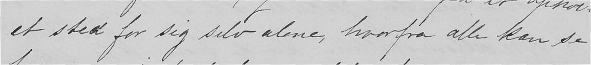et sted for sig selv alene, hvorfra alle kan se
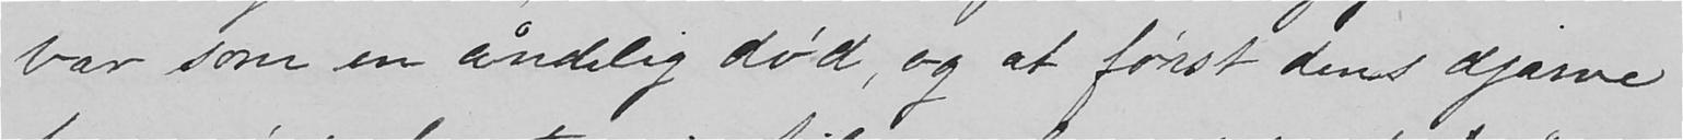var som en åndelig død, og at først dens djerve
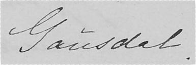Gausdal.
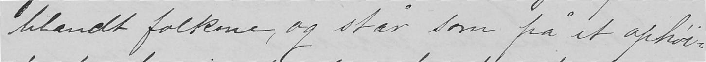blandt folkene, og står som på et ophøi-
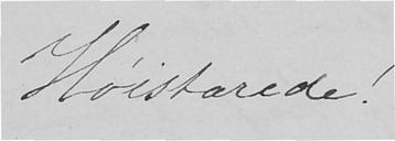Høistærede!
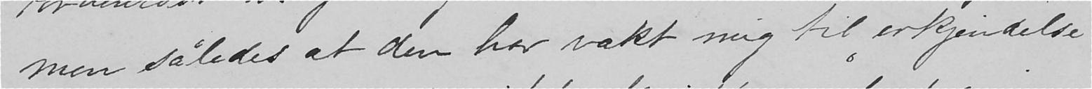Men således at den har vakt mig til erkjendelse
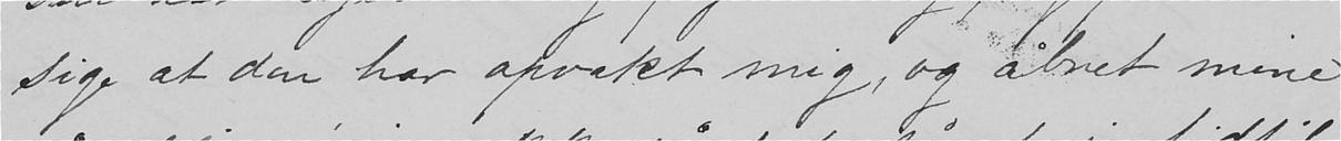sige at den har opvakt mig, og åbnet mine
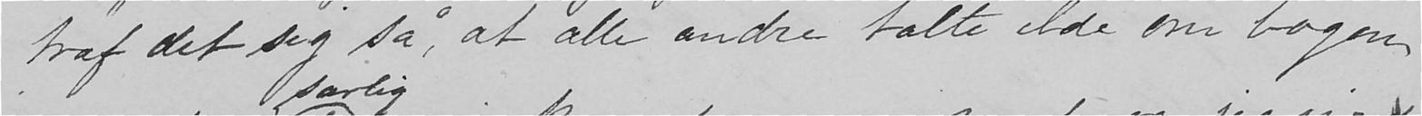traf det sig så, at alle andre talte ilde om bogen
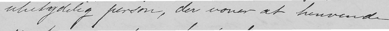ubetydelig person, der vover at henvende
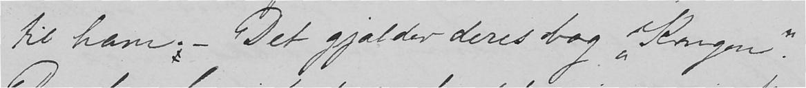til ham. - Det gjelder deres bog "Kongen".
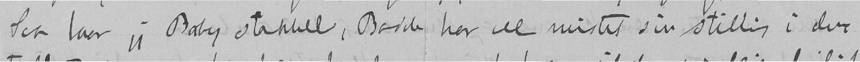Saa har jeg Baby stakkel, Bodde har vel mistet sin stilling i den
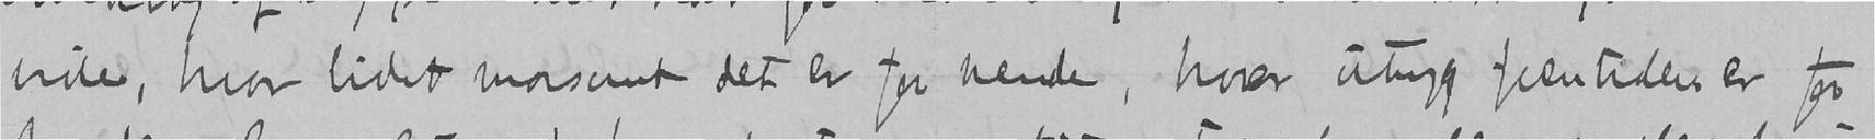vide, hvor lidet morsomt det er for hende, hvor utryg fremtiden er for
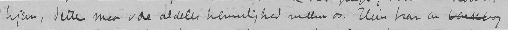hjem, dette maa være aldeles hemmelighed mellem os. Hun har en bonkbog
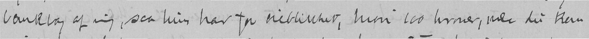bankbog af mig, saa hun har for øieblikket, hvori 100 kroner, men du kan
